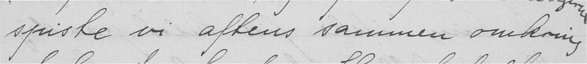spiste vi aftens sammen omkring
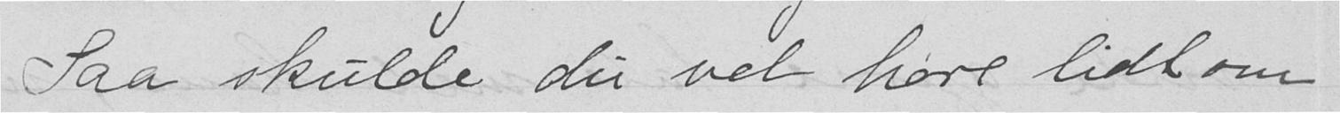Saa skulde du vel høre lidt om
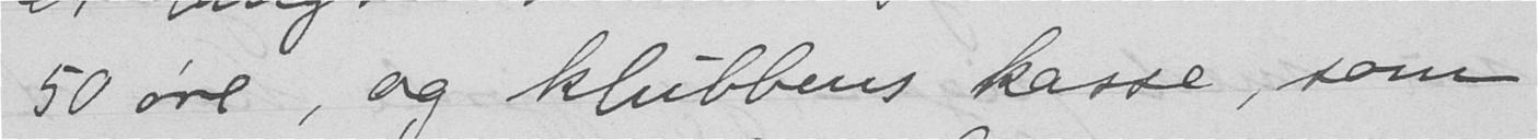50 øre, og klubbens kasse, som
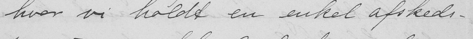hvor vi holdt en enkel afskeds-
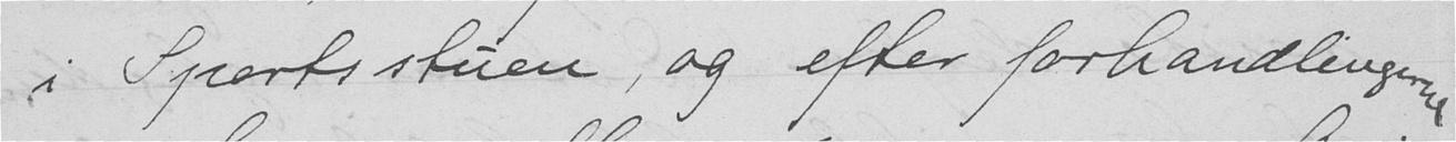i Sportsstuen, og efter forhandlingerne
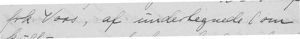frk. Voss, af undertegnede (om
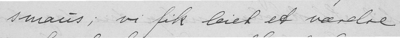smaus; vi fik leiet et værelse
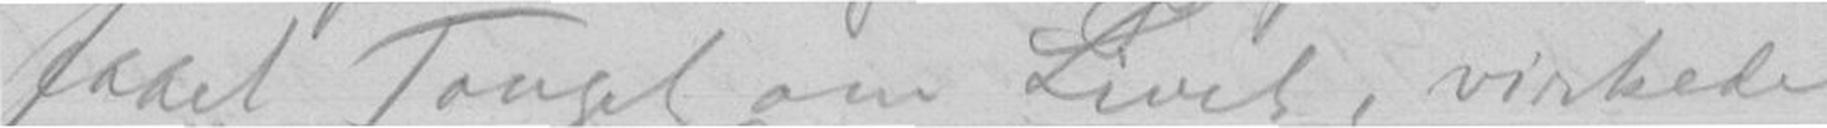faaet Touget om Livet, virkede
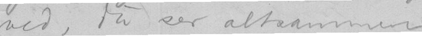ved, du ser altsammen.
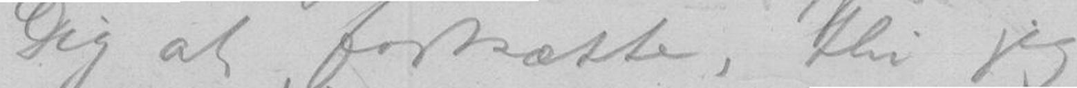Dig at fortsætte, thi jeg
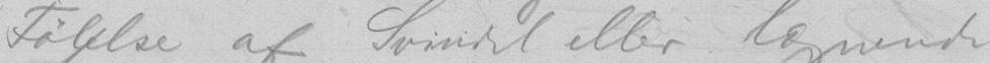Følelse af Svindel eller lignende
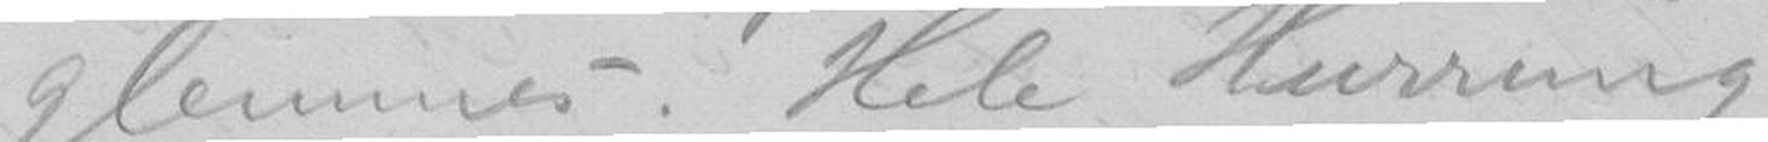glemmes. Hele Hurrung-
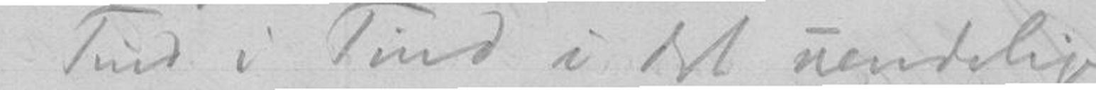og Tind i Tind i det uendelige -
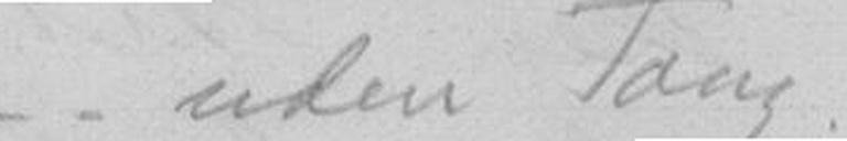- - - - - - - - uden Toug!"
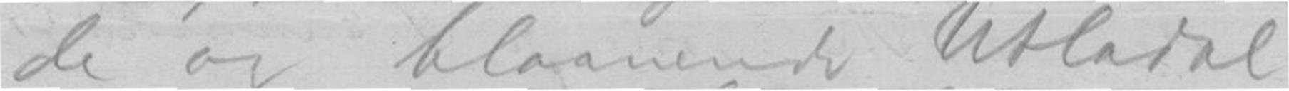de og blaanende Utladal
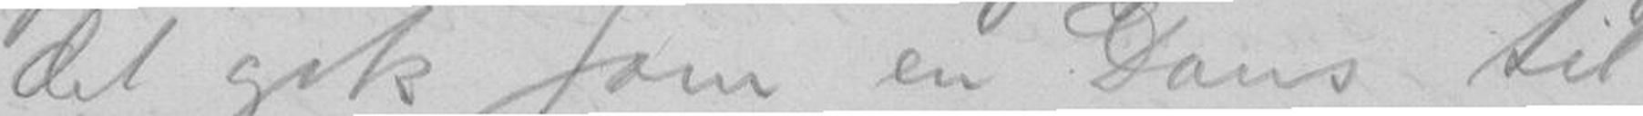det gik som en Dans til
